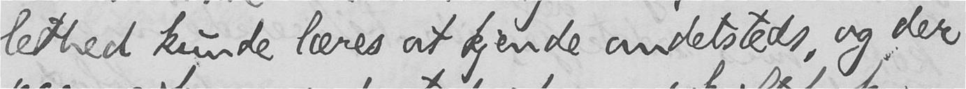lethed kunde læres at kjende andetsteds, og der
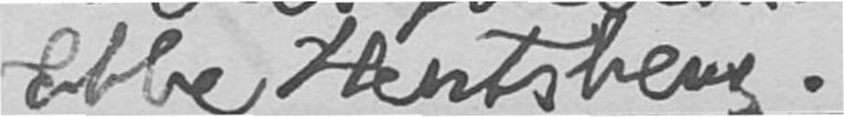Ebbe Hertzberg.
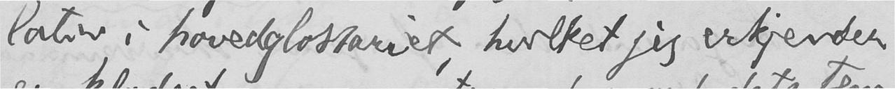lativ i hovedglossariet, hvilket jeg erkjender
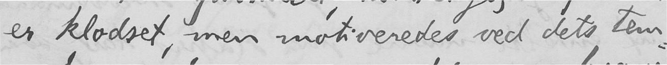er klodset, men motiveredes ved dets tem-
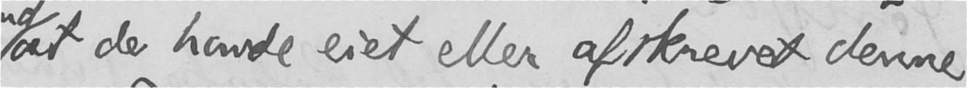at de havde eiet eller afskrevet denne
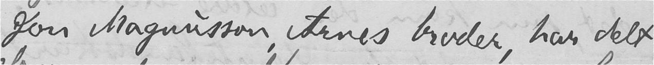Jon Magnusson, Arnes broder, har delt
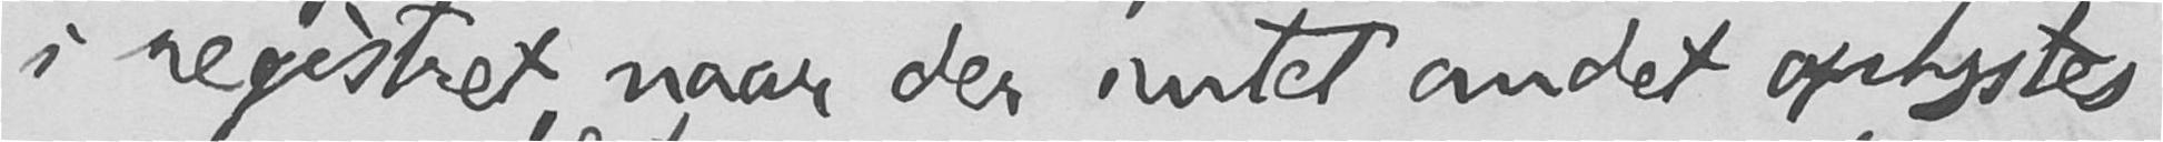i registret, naar der intet andet oplystes
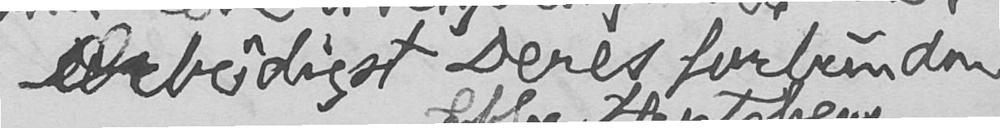Ærbødigst Deres forbundne
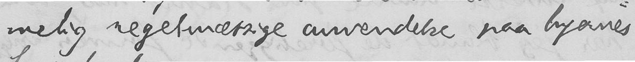melig regelmæssige anvendelse paa byernes
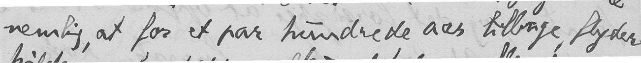nemlig, at for et par hundrede aar tilbage, flyder
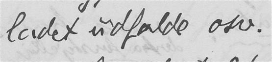ladet udfalde osv.
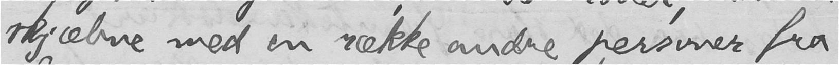skjæbne med en række andre personer fra
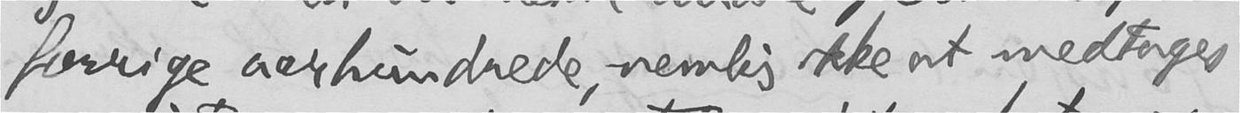forrige aarhundrede, nemlig ikke at medtages
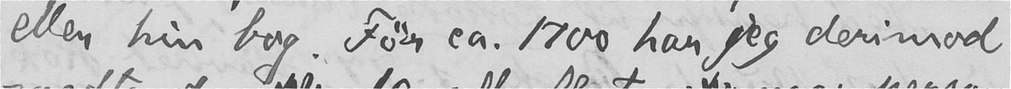eller hin bog. Før ca. 1700 har jeg derimod
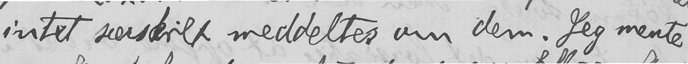intet særskilt meddeltes om dem. Jeg mente
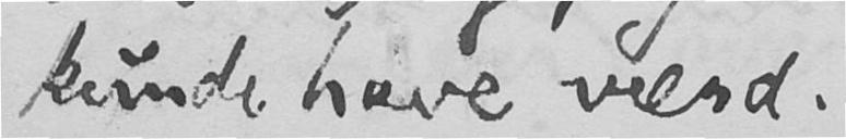kunde have værd.
